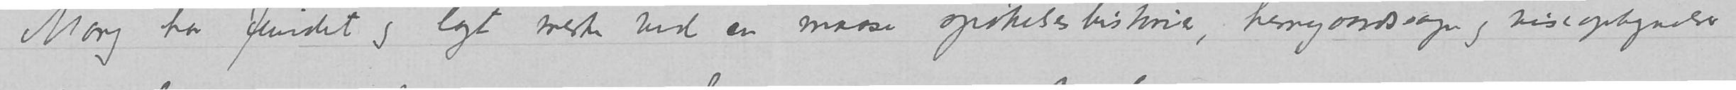Mary har fundet og lagt merke ved en masse spøkelseshistorie, herregaardssagaer og reiseoptegnelser
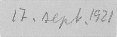17. sept. 1921.
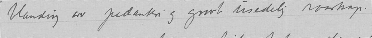blanding av pedanteri og grovt usedelig raaskap.
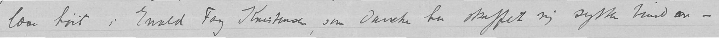læse høit i Evald Tang Kristensen, som Dancke har skaffet mig sytten bind av –
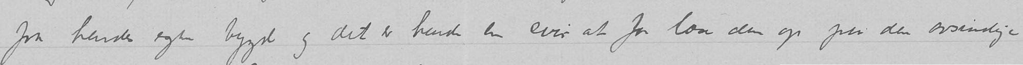fra hendes egen bygd og det er hende en svir at for læse dem op paa den avsindige
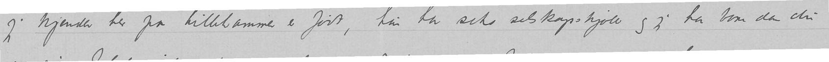jeg kjender her paa Lillehammer er født, hun har sike selskapskjoler og jeg har bare den du
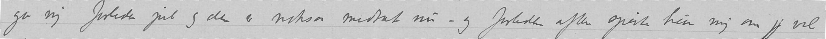go mig forlede jul og den er noksaa medtat nu – og forleden aften spurte hun mig om jeg vel
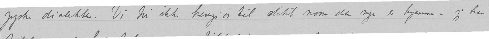jyske dialekten. Vi tør ikke hengi os til slikt naar den nye er hjemme – jeg har
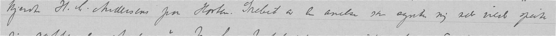kjendte H.C. Andersens paa Horten. Grebet av en anelse som syntes mig selv vild spurte
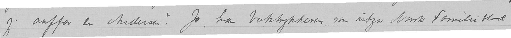jeg aaffer en Andersen! Jo, han boktrykkeren, som utgav Norsk Familieblad
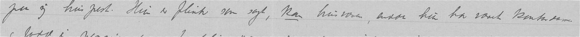paa sig huspost. Hun er flink som sagt, kan husværen, endda hun har været kontordame
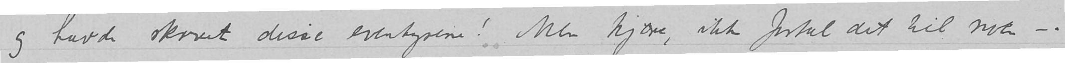og hadde skrevet disse eventyrine! Men kjære, ikke fortel det til noen –.
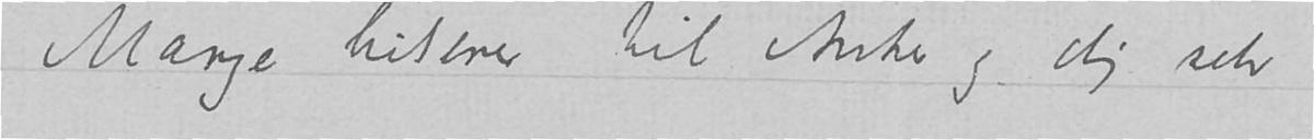Mange hilsener til Anker og dig selv
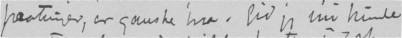paatvinger, er ganske bra. Gid jeg nu kunde
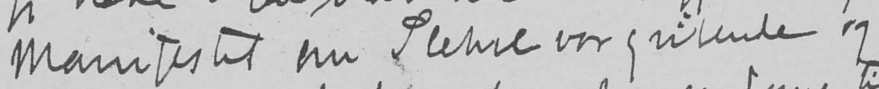Manifestet om Plekre var gribende og
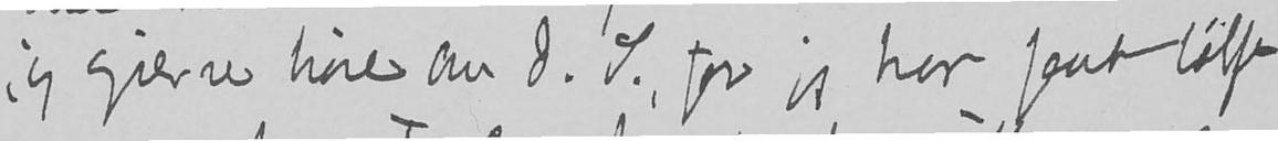jeg gierne høre om O. S., for jeg har faat løfte
